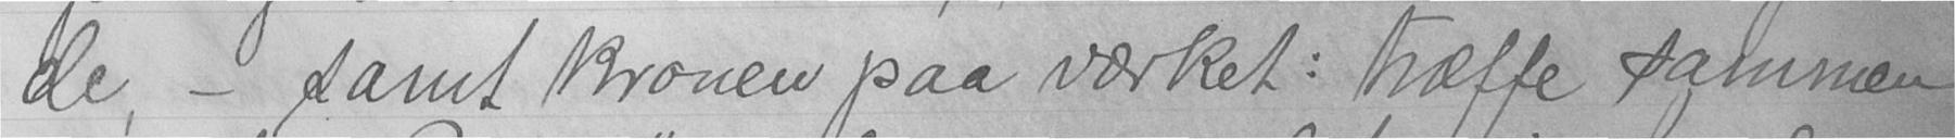de, - samt kronen paa værket: tæffe sammen
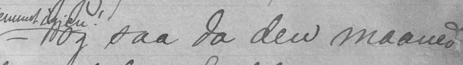- sog saa da den maaned
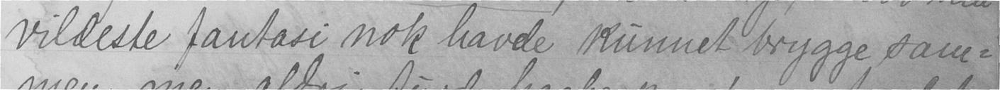vildeste fantasi nok havde kunnet brygge sam-
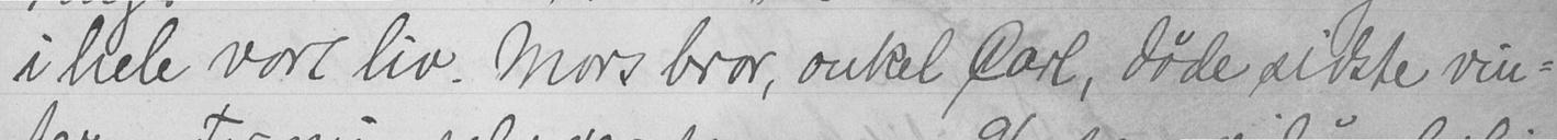i hele vort liv. Mors bror, onkel Carl, døde sidste vin-
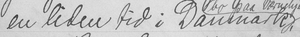en liden tid i Danmark,
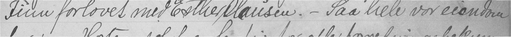Finn forlovet med Esther Clausen. - Saa hele vor eiendom
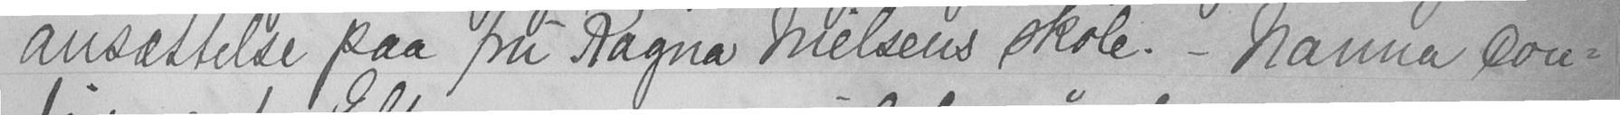ansættelse paa fru Ragna Nielsens skole. - Nanna con-
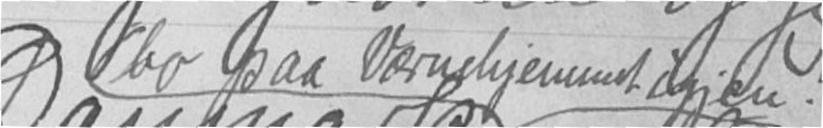bo paa Værnehjemmet igjen!
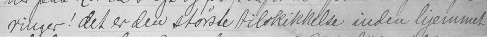ringer! det er den største tilskikkelse inden hjemmet
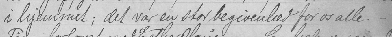i hjemmet; det var en stor begivenhed for os alle. -
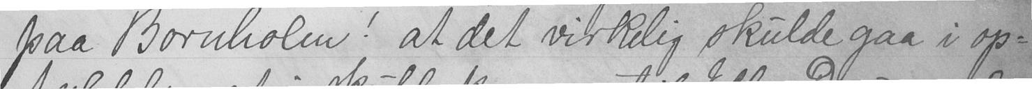paa Bornholm! at det virkelig skulde gaa i op-
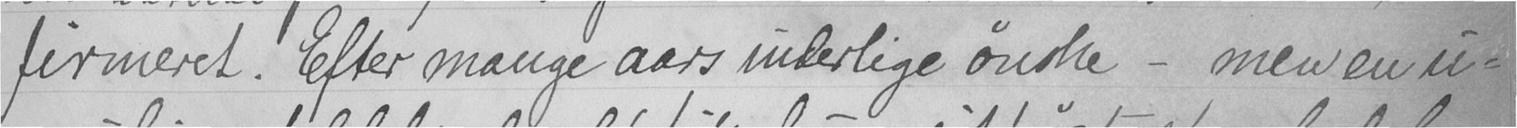firmeret. Efter mange aars inderlige ønske - men en u-
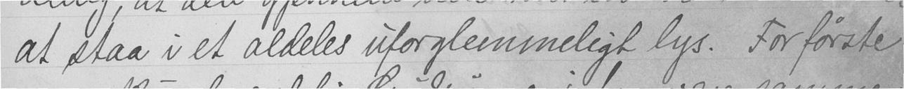at staa i et aldeles uforglemmeligt lys. For første
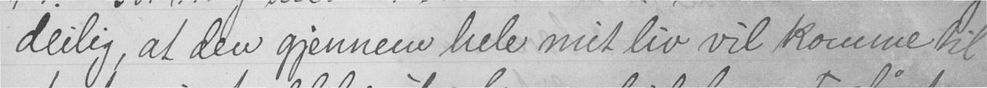deilig, at den gjennem hele mit liv vil komme til
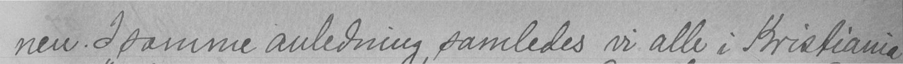nen. I samme anledning samledes vi alle i Kristiania
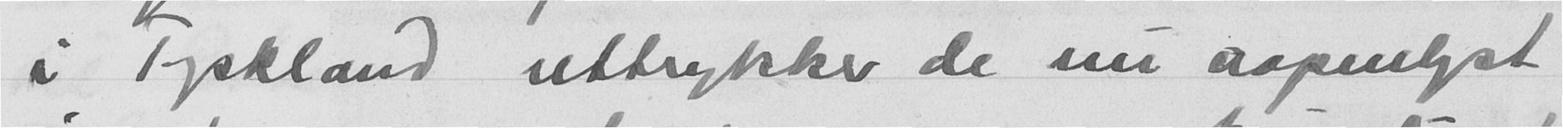i Tyskland uttrykker de nu aapenlyst
